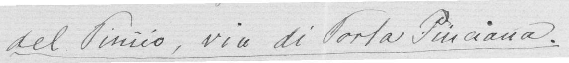del Pincio, via di Porta Pinciana.
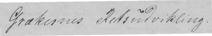Grækernes Retsudvikling.
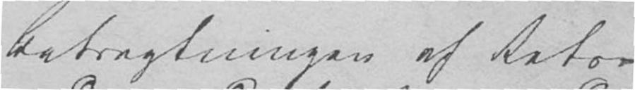Betragtningen af Rets-
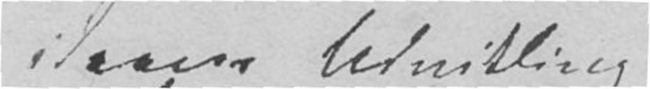ideenes Udvikling
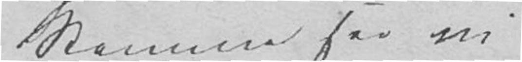Stamme ser vi
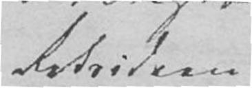Retsideen
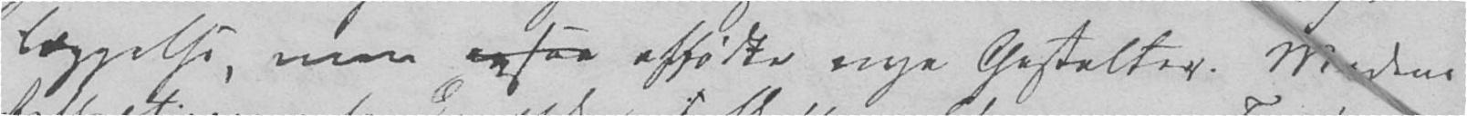læggelse, men ogsaa affødte nye Gestalter. Medens
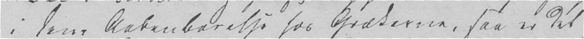i dens Aabenbarelse hos Grækerne, saa er det
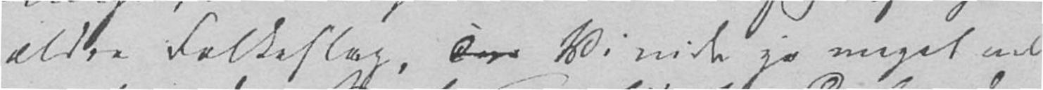ældre Folkeslag. Vi vide jo meget vel
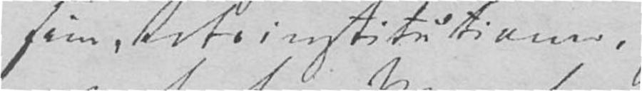som Retsinstitutioner,
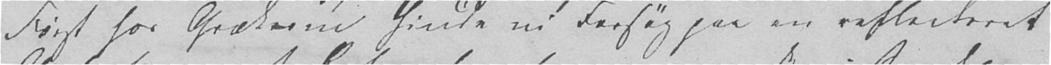Først hos Grækerne finde vi Forsøg paa en reflecteret
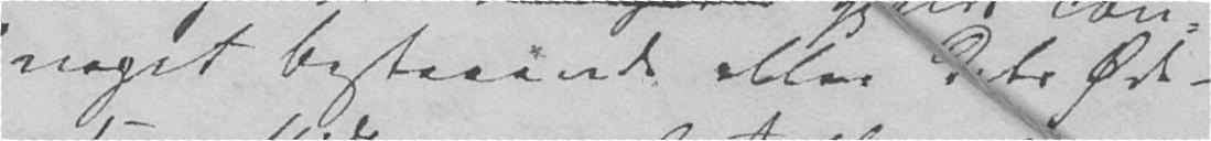noget bestaaende eller dets Øde-
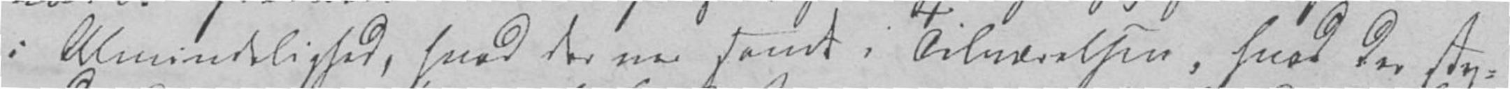i Almindelighed, hvad der var sandt i Tilværelsen, hvad der sty-
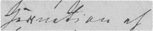servation af
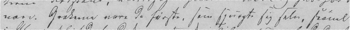vare. Grækene vare de første, som spurgte sig selv, saavel
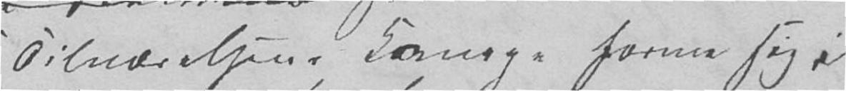Tilværelsens Konge forme sig i
